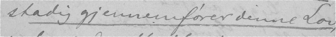stadig gjennemfører denne Lov,
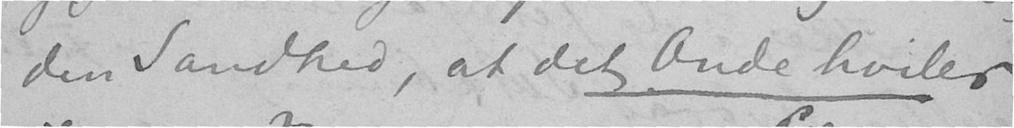den Sandhed, at det Onde hviler
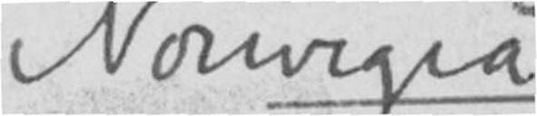Norwegia
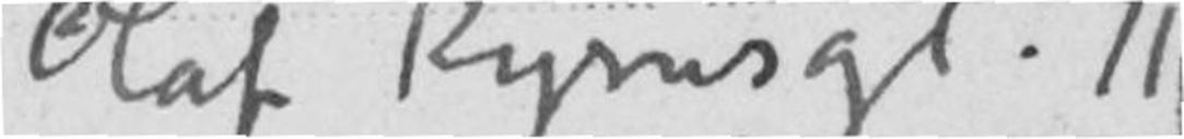Olaf Kyrresgd. 11
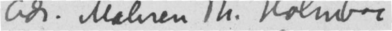Adr. Maleren Th. Holmboe
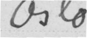Oslo
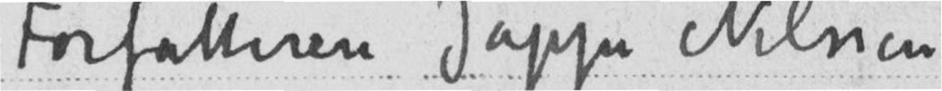Forfatteren Jappe Nilssen
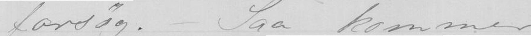forsøg. - Saa kommer
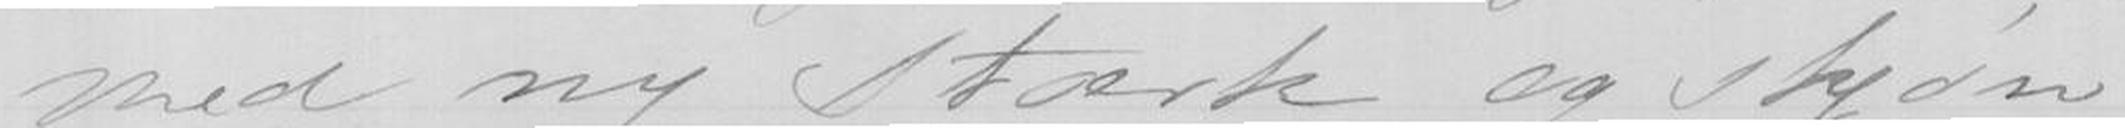med ny stærk og skjøn
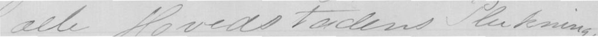alle Hovedstadens Pluknings-
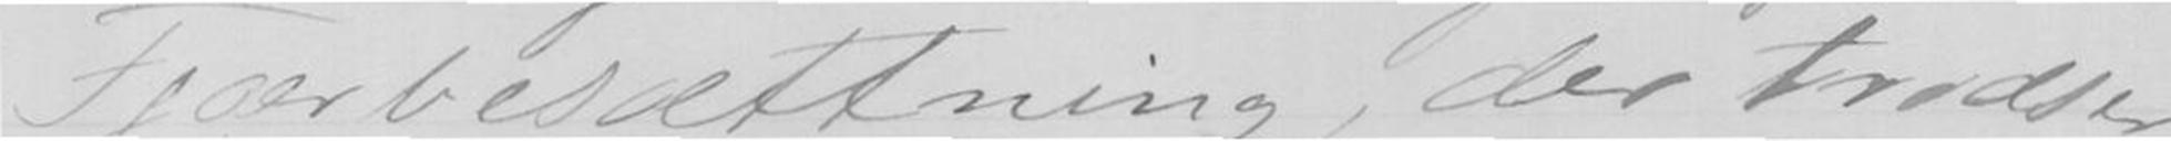Fjærbesættning, der trodser
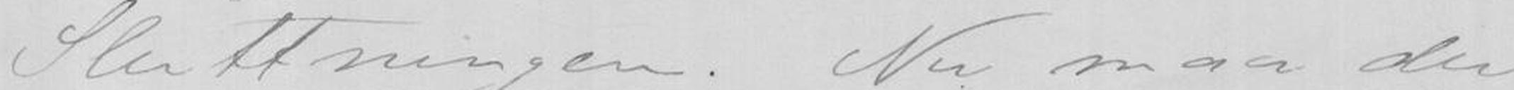Sluttningen. Nu maa du
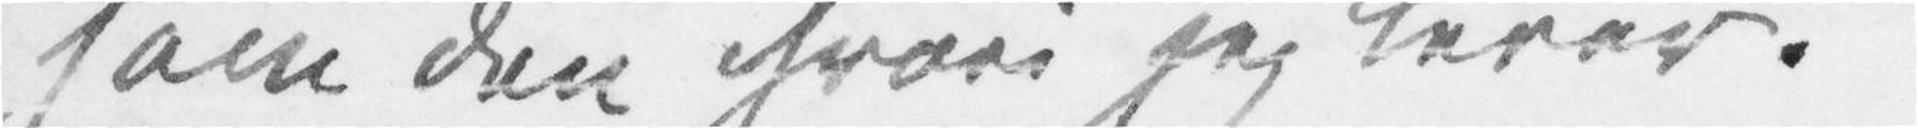som den hvori jeg lever.
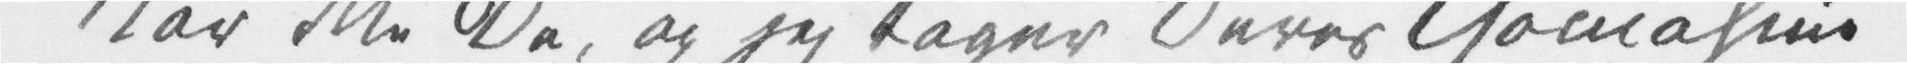Når ikke De, og jeg tager Deres Thomasine
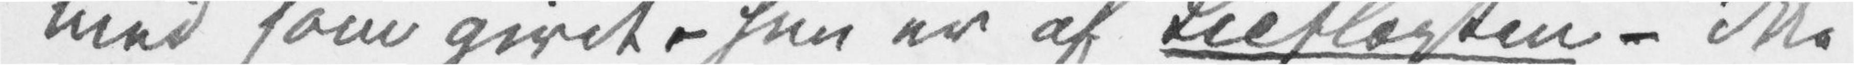med som givet – hun er af Lieslægten – ikke
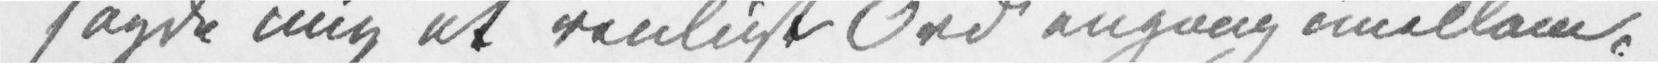sagde mig et venligt Ord engang imellom,
